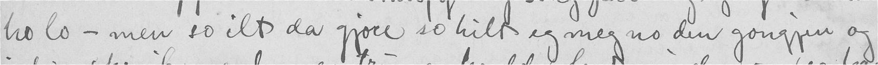ho lo, - men so ilt da gjore so hilt eg meg no den gongjen og
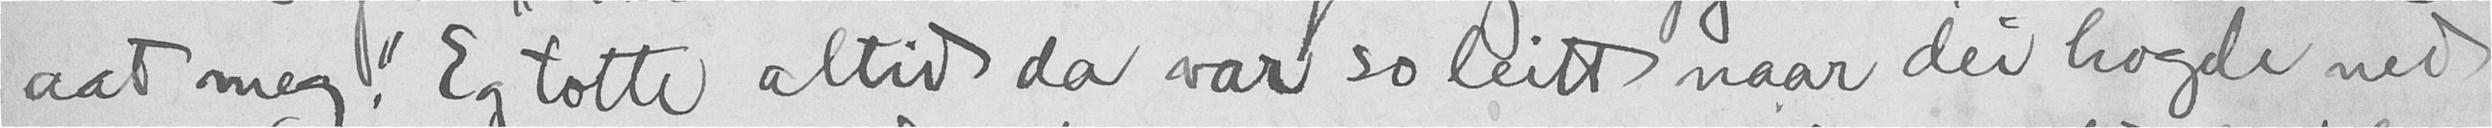aat mig." Eg totte altid da var so leitt naar dei hogde ned
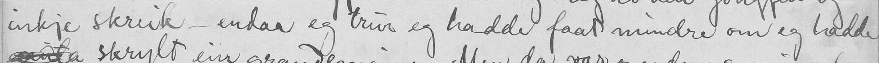inkje skreik - endaa eg trur og hadde faat mindre om eg hadde
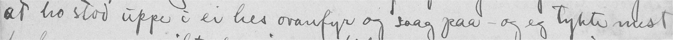at ho stod uppe i ei hes ovanfyr og saag paa - og eg tykte mest
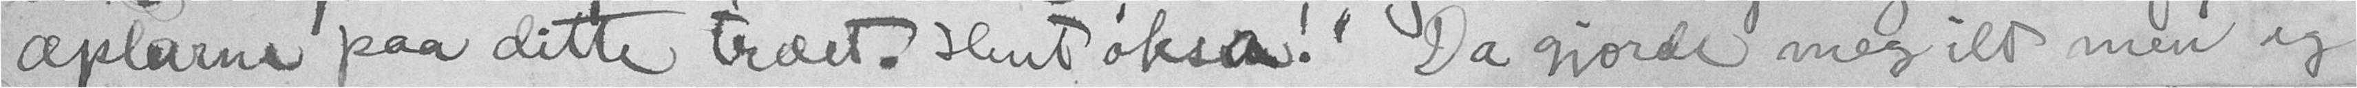æplarna paa ditte træet. Hent øksa!" Da gjorde meg ilt men eg
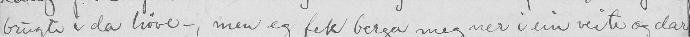brugte i da høve -, men eg fek berga meg ner i ein veite og der
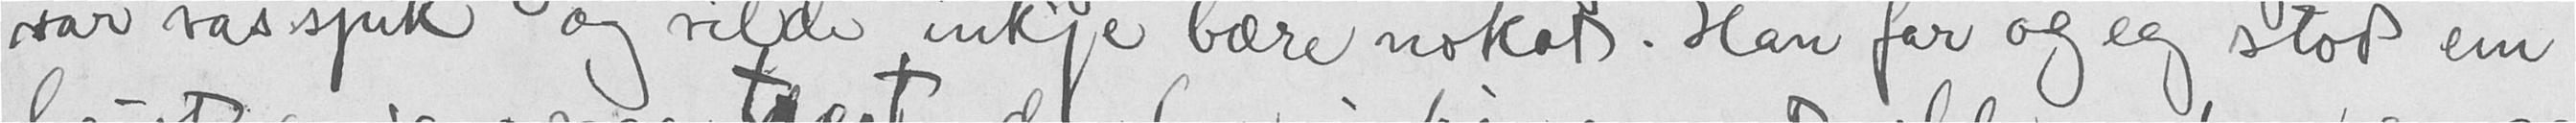var vassjuk og vilde inkje bære nokot. Han far og eg stod ein
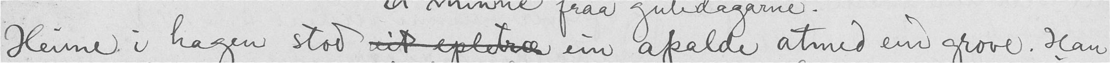Heime i hagen stod  ein apalde atmed ein grove. Han
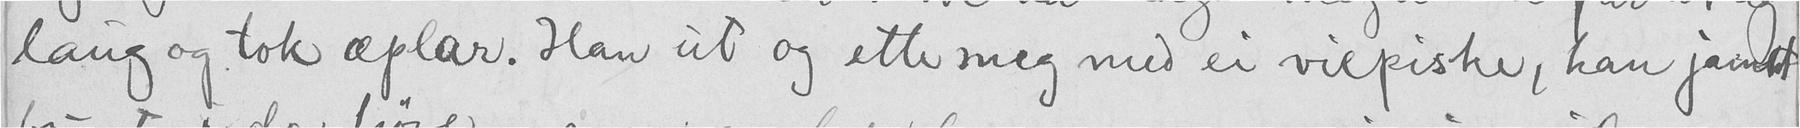laug og tok æplar. Han ut og ette meg med ei vilpiske, han jamt
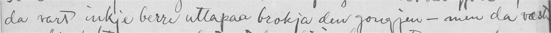da vart inkje berre uttapaa brokja den gongjen, - men da væste
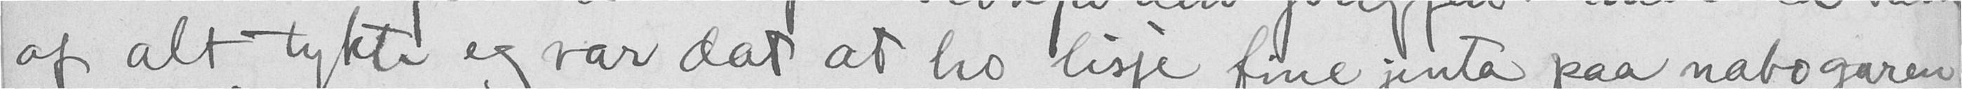af alt tykte eg var dat at ho lisje fine jenta paa nabogaren
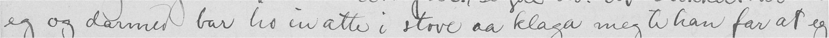eg og dermed bar ho in atte i stove aa klaga meg te han far at eg
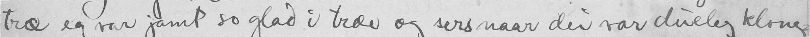træ og var jamt so glad i træe og sers naar der var duelig klong
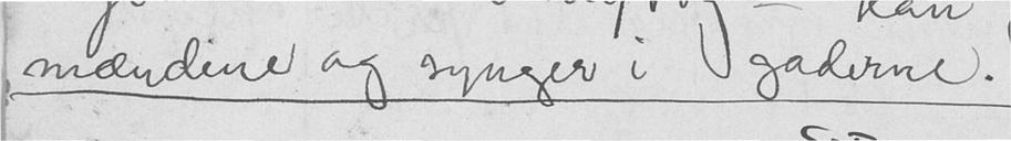mændene og synger i gaderne.
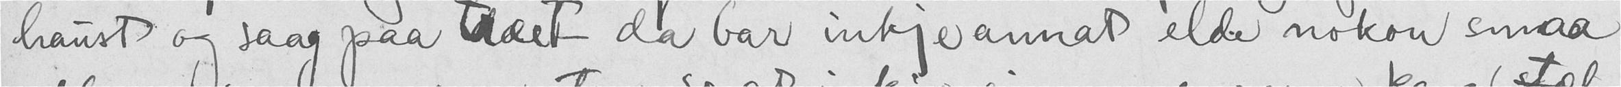haust og saag paa træet da bar inkje annat elde nokon smaa
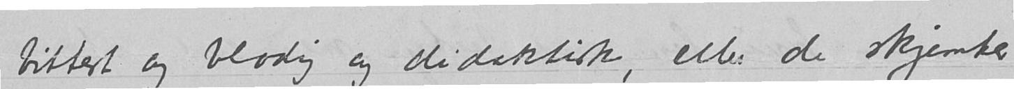bittert og blodig og didaktisk, eller de skjemter
